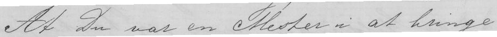At Du var en Mester i at bringe
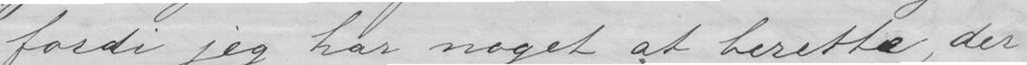fordi jeg har noget at berette, der
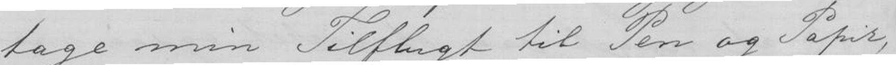tage min Tilflugt til Pen og Papir,
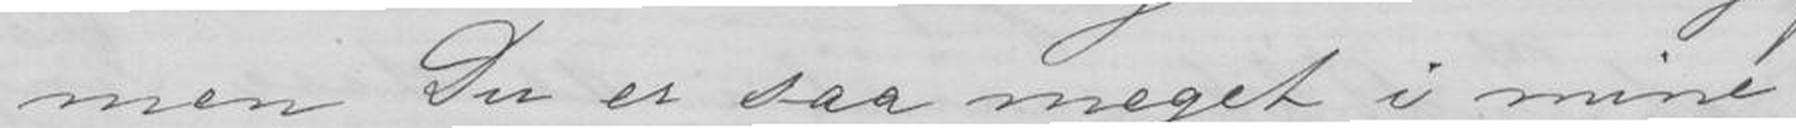men Du er saa meget i mine
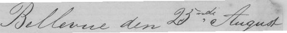Bellevue den 25nde August
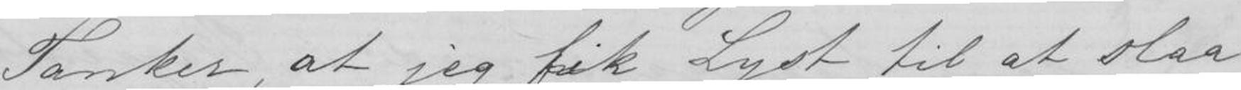Tanker, at jeg fik Lyst til at slaa
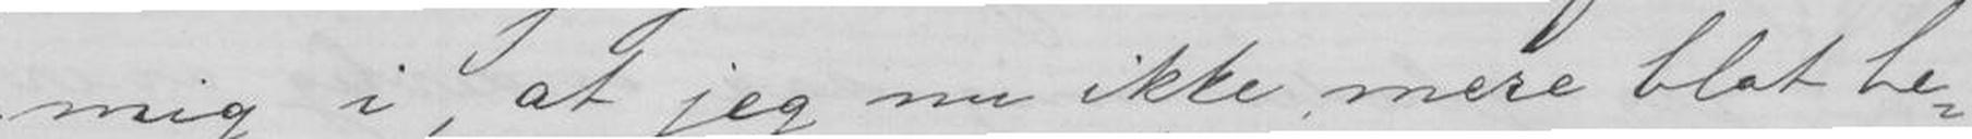mig i, at jeg nu ikke mere blot be-
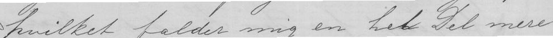hvilket falder mig en hel Del mere
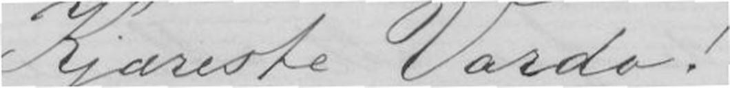Kjæreste Vardo!
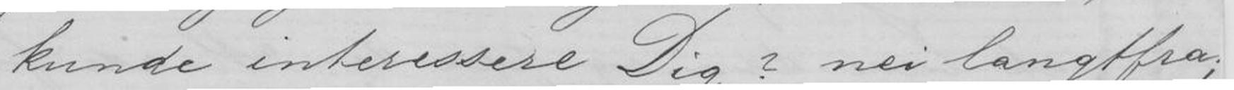kunde interessere Dig? nei langtfra;
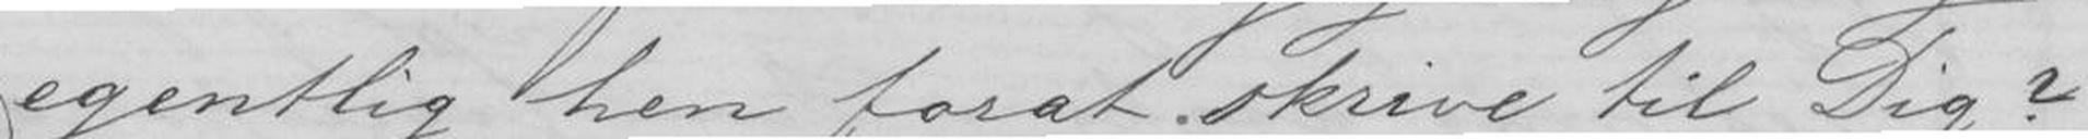egentlig hen forat skrive til Dig?
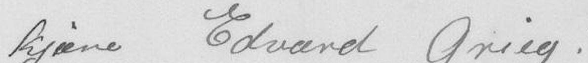Kjære Edvard Grieg!
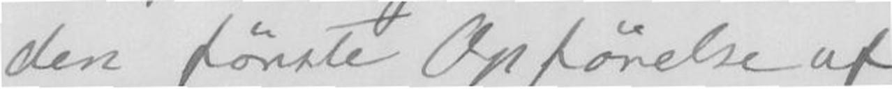den første Opførelse af
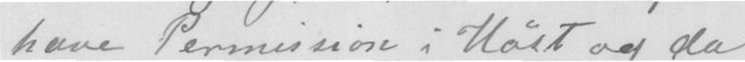have Permission i Høst og da
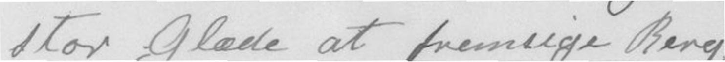stor Glæde at fremsige Berg-
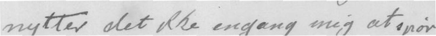nytter det ikke engang mig at spør-
